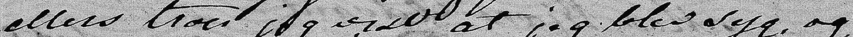ellers troer jeg vist at jeg blev syg, og
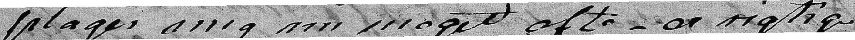plager mig nu meget ofte, er rigtig-
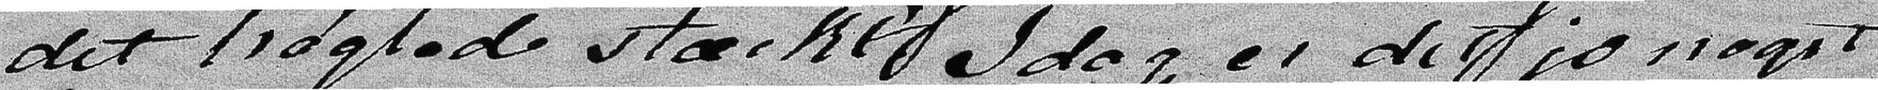det haglede Stærkt. Idag er det jo noget
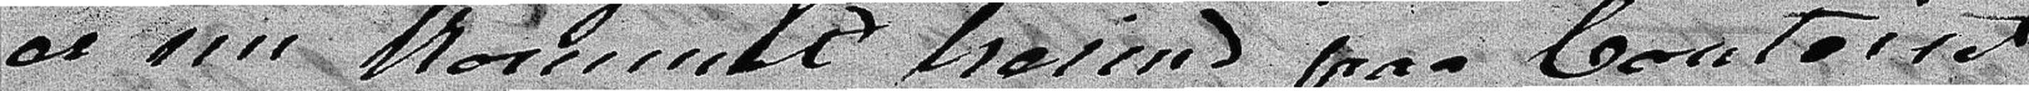er nu kommet herind paa Contoiret for
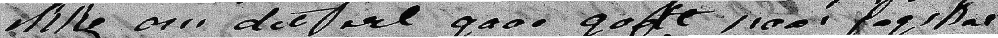ikke om det vil gaae godt naar jeg skal
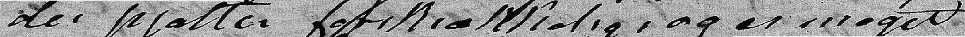der pjatter forskrækkelig; og er meget
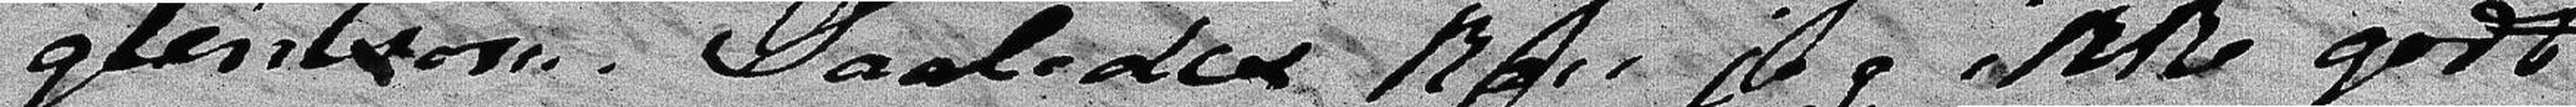glemsom. Saaledes kan jeg ikke godt
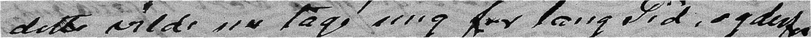dette vilde nu tage mig for lang Tid, og derfor
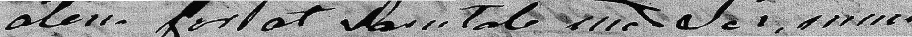alene for at samtale med Jer, mine to
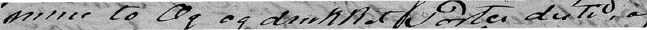mine to Æg og drukket Porter dertil, og
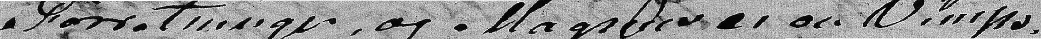Forretninger, og Magnus en Vimps
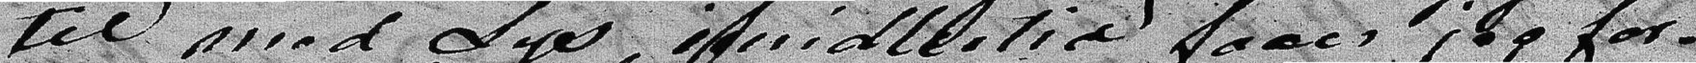til med Lys, imidlertid faaer jeg for-
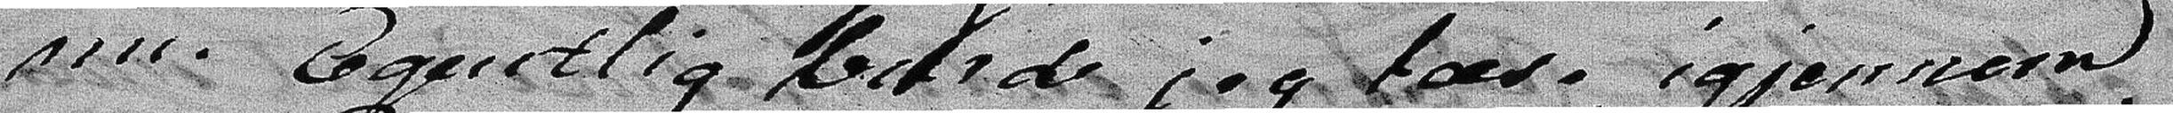nu. Egentlig burde jeg læse igjennem
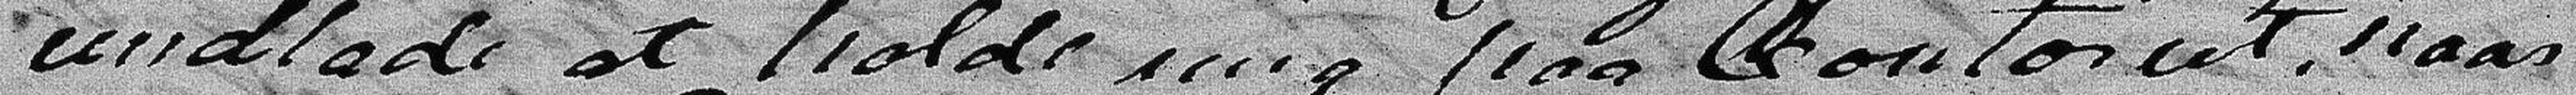undlade at holde mig paa Contoiret, naar
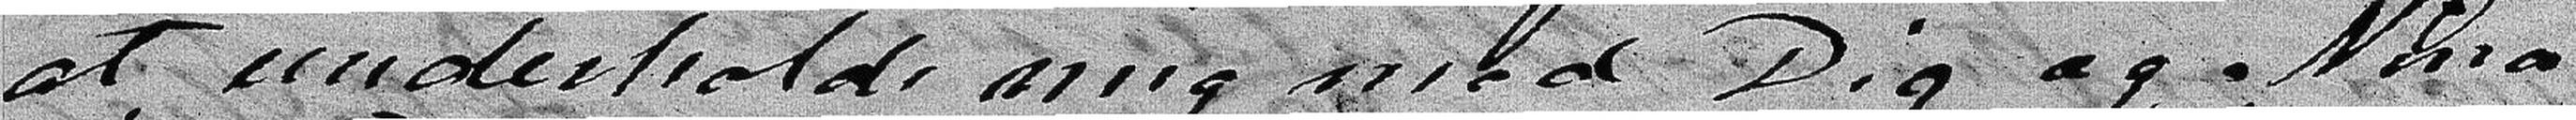at underholde mig med Dig og Nina
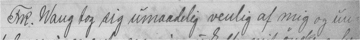Frk. Wang tog sig umaadelig venlig af mig og un-
